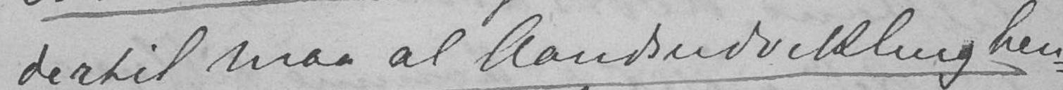dertil maa al Aandsudvikling hen-
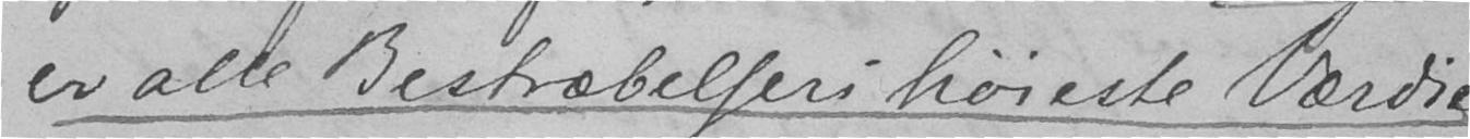er alle Bestræbelsers høieste Værdie,
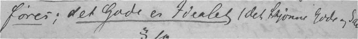føres; det Gode er Idealet (det skjønne Gode og Sande)
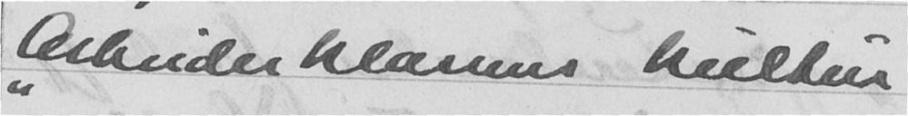"Arbeiderklassens kultur
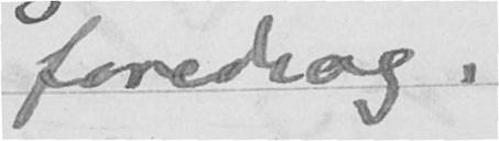foredrag.
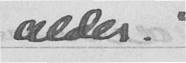alder."
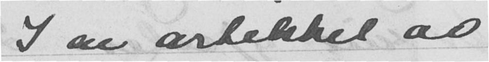I en artikkel av
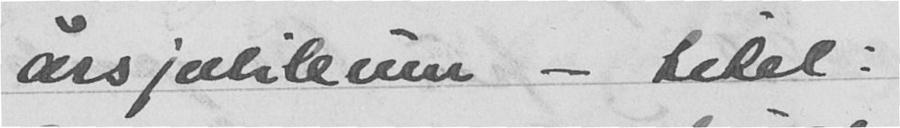årsjubileum - tilel:
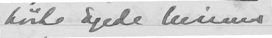og hørte Egede Nissens
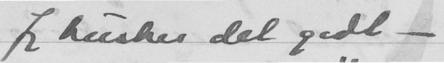Jeg husker det godt -
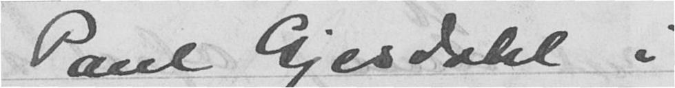Paul Gjesdahl i
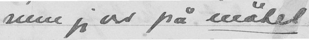men jeg var på møtet
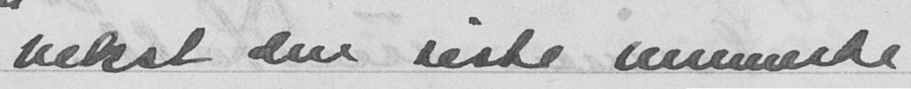vekst den siste menneske
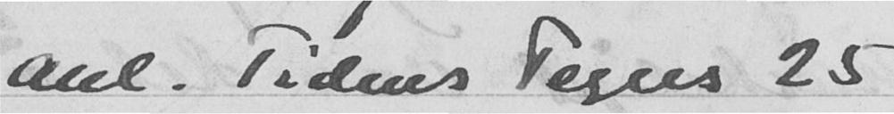anl. Tidens Tegns 25
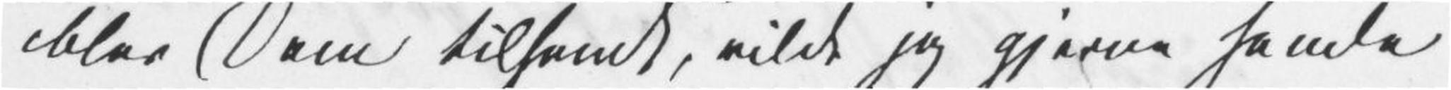blev Dem tilsendt, vilde jeg gjerne samle
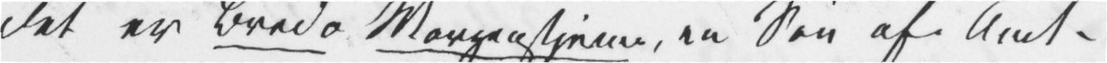det er Bredo Morgenstjerne, en Søn af Amt=
